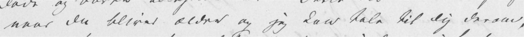naar Du bliver ældre og jeg kan tale til Dig derom,
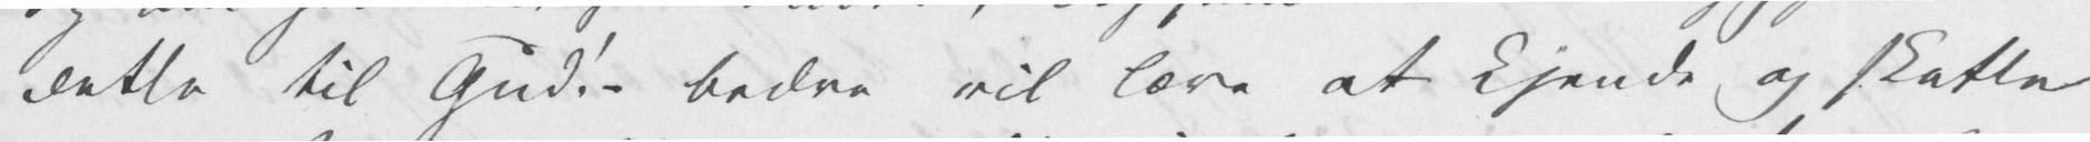dette til Gud! – bedre vil lære at kjende og skatte
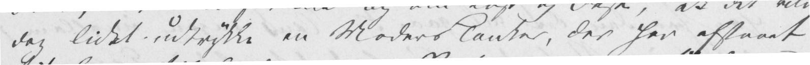dog lidet udtrykke en Moders Tanker, der har afstaaet
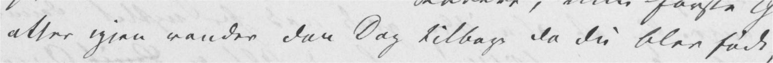atter igjen vender den Dag tilbage da Du blev født,
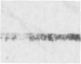Lillehammer
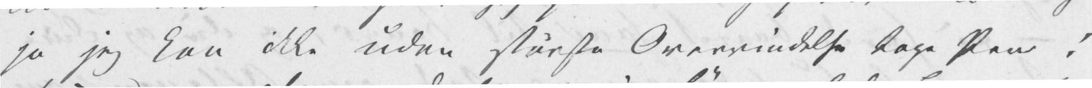ja jeg kan ikke uden største Overvindelse tage Pen i
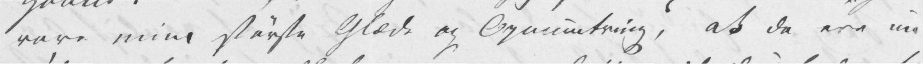vare min største Glæde og Opmuntring, ak de ere nu
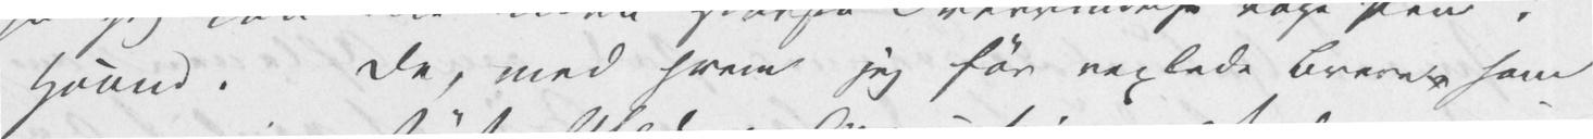Haand. De, med hvem jeg før vexlede Breve, som
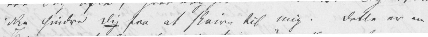ikke hindre Dig fra at skrive til mig. Dette er en
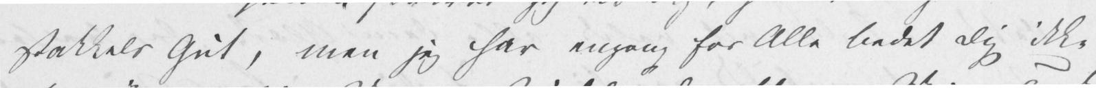stakkels Gut, men jeg har engang for Alle bedet Dig ikke
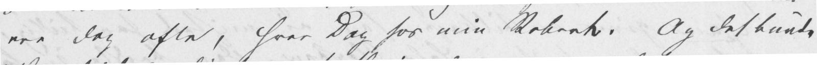ere dog ofte, hver Dag hos min Robert. Og det kunde
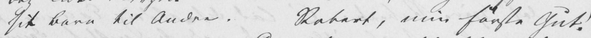sit Barn til Andre. Robert, min første Gut!
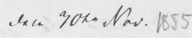Den 30te Nov. Collett
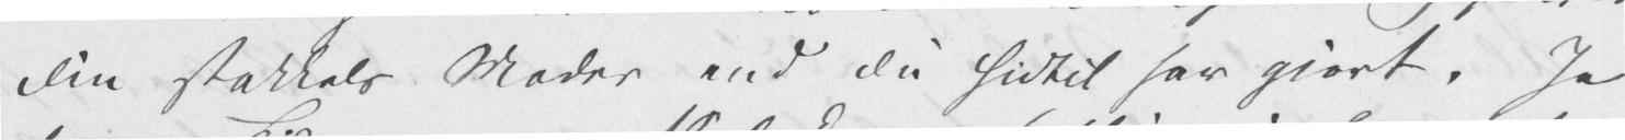Din stakkels Moder end Du hidtil har gjort. Ja
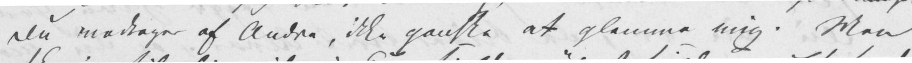Du modtager af Andre, ikke ganske at glemme mig. Men
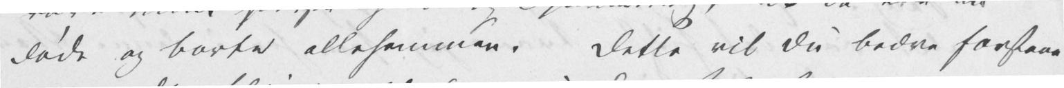døde og borte allesammen. Dette vil Du bedre forstaae
